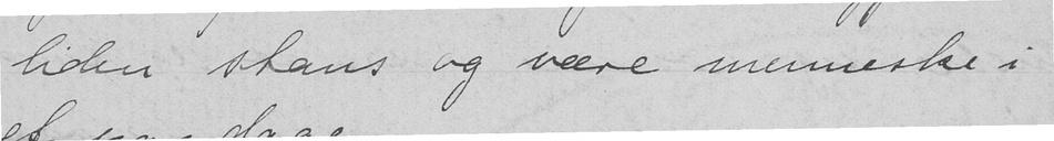liden stans og være mennske i
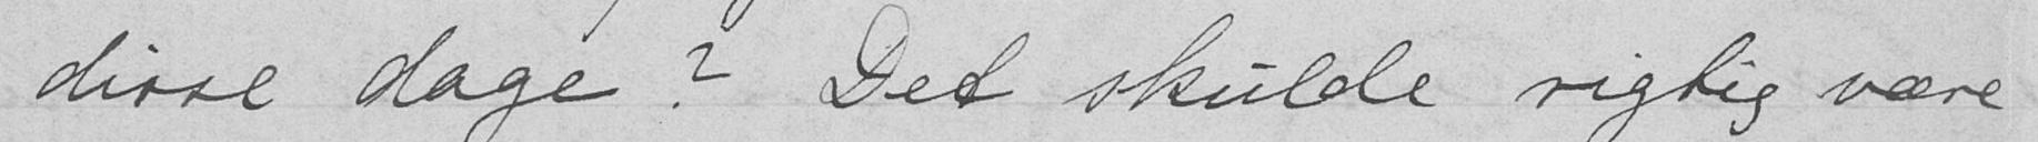disse dage? Det skulde rigtig være
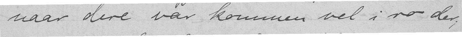naar dere var kommen vel i ro der;
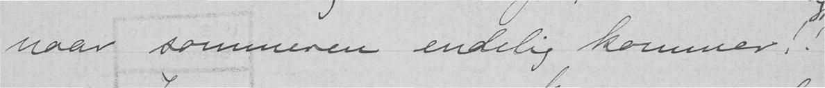naar sommeren endelig kommer!!
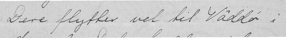Dere flytter vel til Väddø i
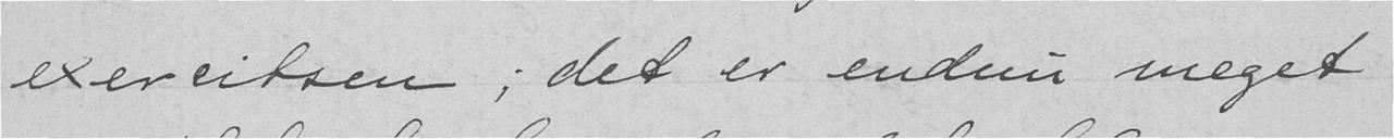exercitsen; det er endnu meget
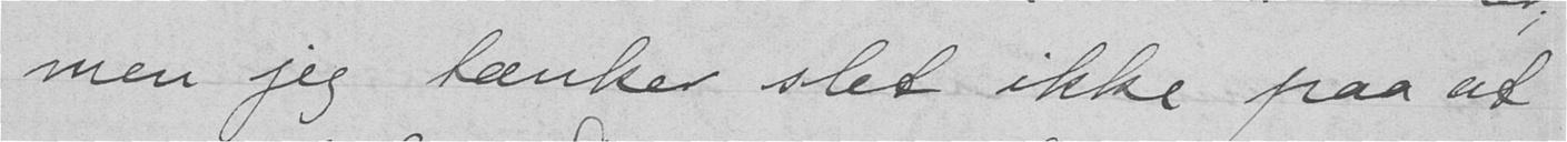men jeg tænker slet ikke paa at
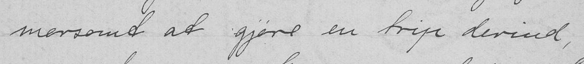morsomt at gjøre en trip derind,
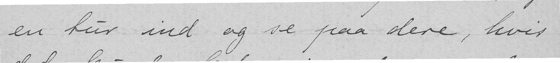en tur ind og se paa dere, hvis
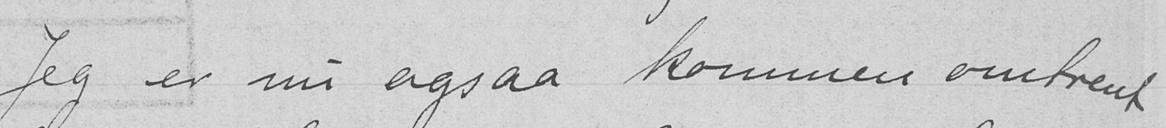Jeg er nu ogsaa kommen omtrent
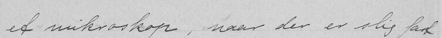et mikroskop, naar der er slig fart
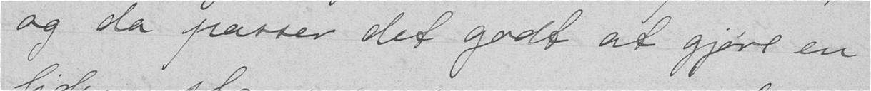og da passer det godt at gjøre en
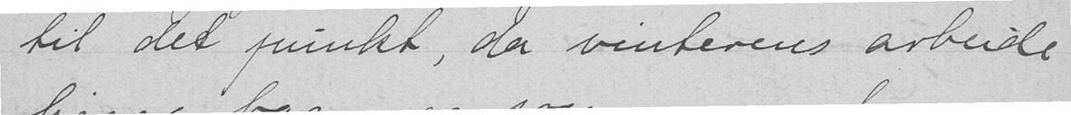til det punkt, da vinterens arbeide
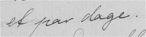et par dage.
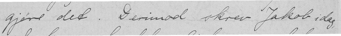gjøre det. Derimod skrev Jakob i dag,
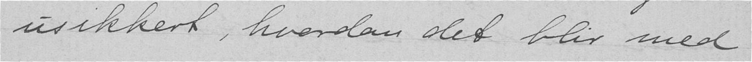usikkert, hvordan det blir med
